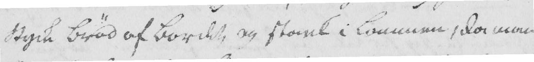stycke Brød af Bordet og stak i Lommen, da man
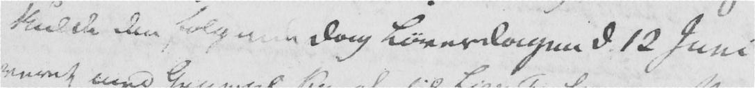Kulde den følgende Dag Løverdagen d 12 Juni
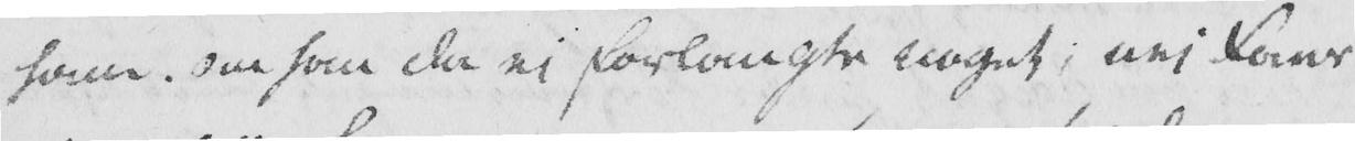ham. Om han da ei forlangte noget; nej Faer
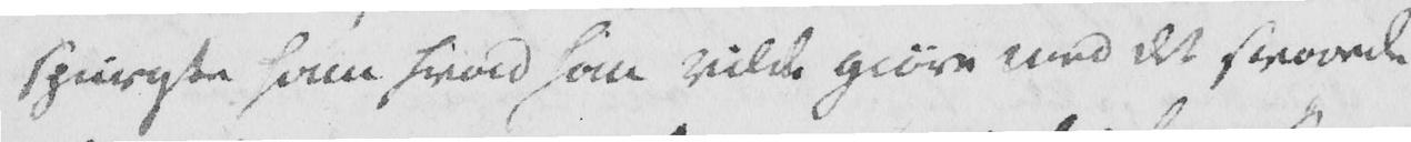spurgte ham hvad han vilde giøre med det svarede
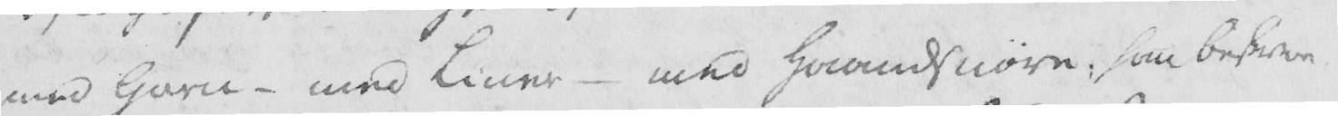med Garn - med Liner - med Haandsnøre, som beskrevne.
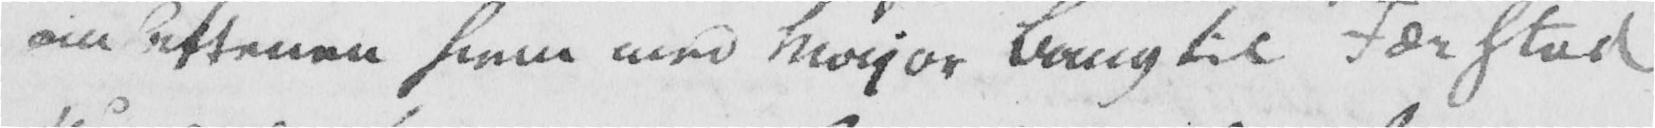om Aftenen hiem med Major Bang til Færstad.
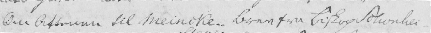Om Aftenen til Meincke. Brev fra Biskop Schønhei-
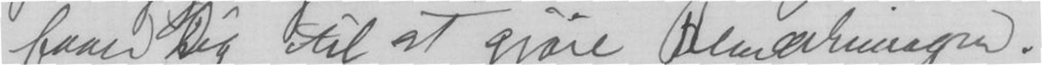faaer Dig til at gjøre Bemærkninger
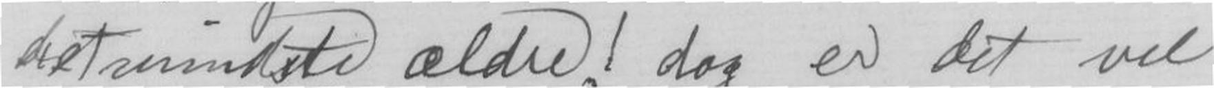detmindste ældre! dog er det vel
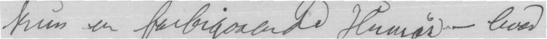kun en forbigaaende Humør - hvad
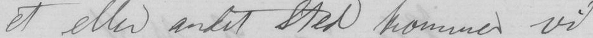et eller andet sted kommer vi
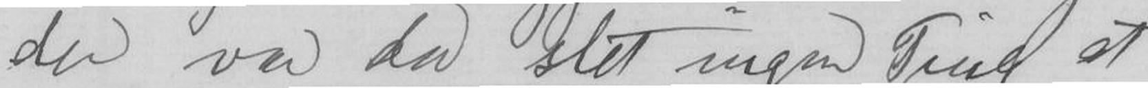der var da slet ingen Ting at
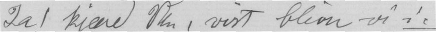Ja! kjære Ven, vist bliver vii-
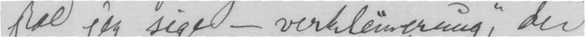skal jeg sige - verkleinerung, der
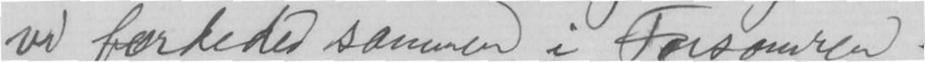vi færdedes sammen i Forsomren.
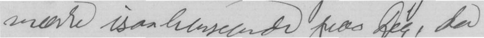snakke isaahenseende paa Dig, da
